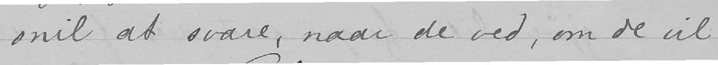snil at svare, naar de ved, om de vil
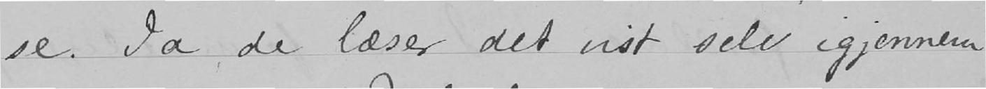se. Ja de læser det vist selv igjennem
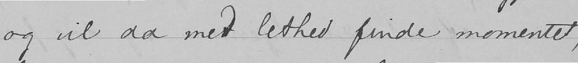og vil da med lethed finde momentet,
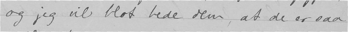og jeg vil blot bede dem, at de er saa
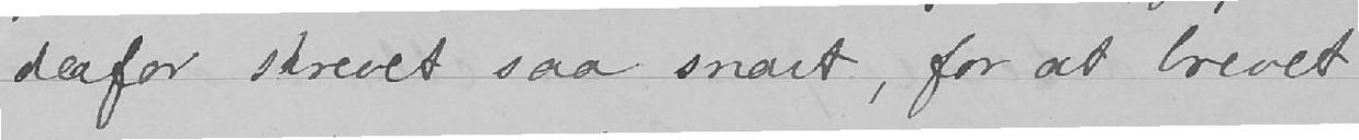derfor skrevet saa snart, for at brevet
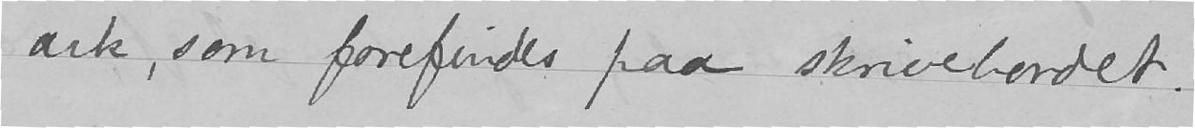ark, som forefindes paa skrivebordet.
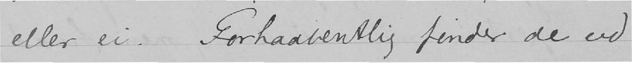eller ei. Forhaabentlig finder de ud
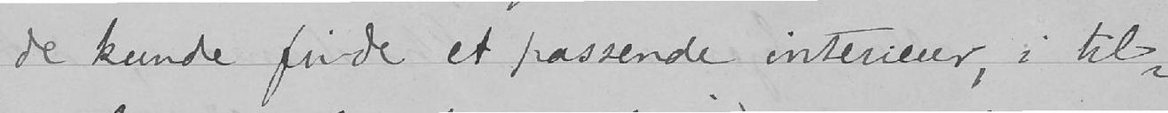de kunde finde et passende interieur, i til-
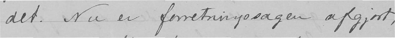det. Nu er forretningssagen afgjort,
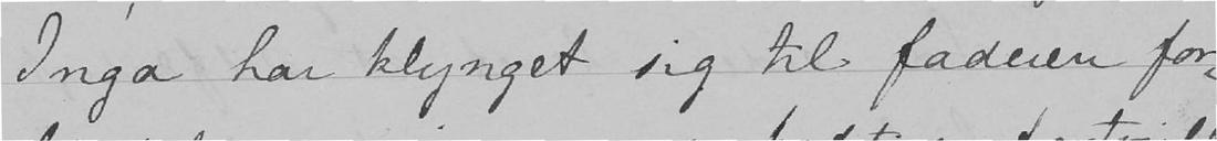Inga har klynget sig til faderen for-
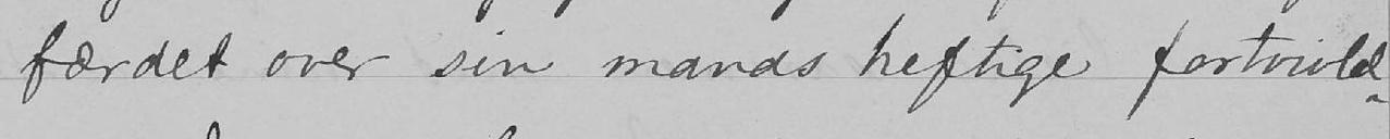færdet over sin mands heftige fortvivlel-
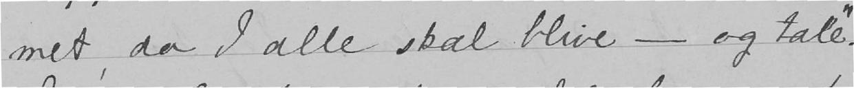met, da I alle skal blive – og tale».
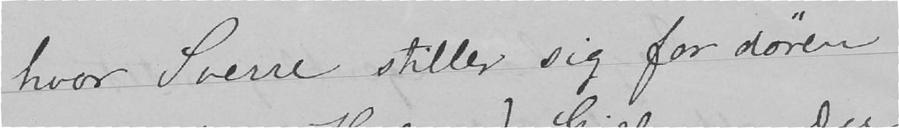hvor Sverre stiller sig for døren
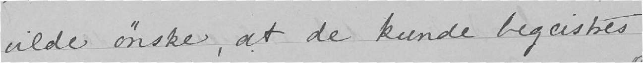vilde ønske, at de kunde begeistres
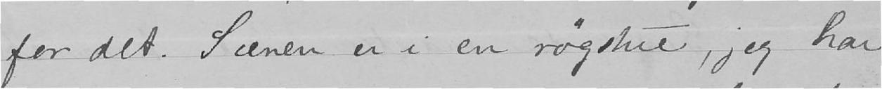for det. Scenen er i en røgstue, jeg har
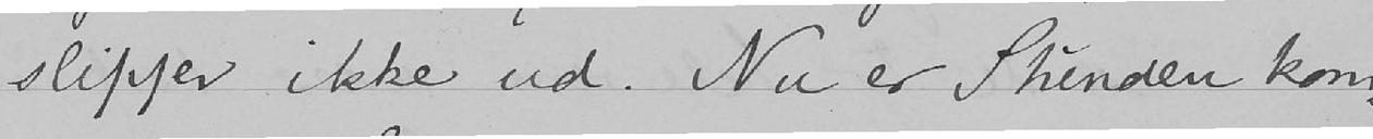slipper ikke ud. Nu er Stunden kom-
